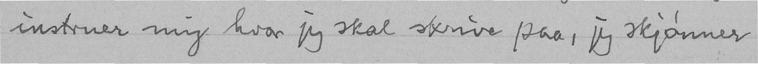instruer mig hvor jeg skal skrive paa, jeg skjønner
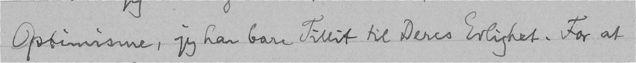Optimisme, jeg har bare Tillit til Deres Erlighet. For at
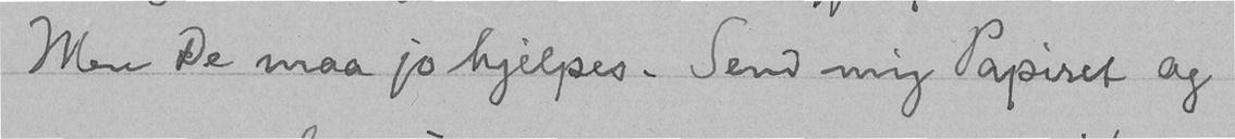Men De maa jo hjelpes. Send mig Papiret og
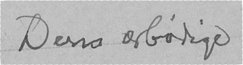Deres ærbødige
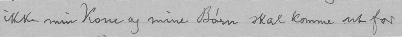ikke min Kone og mine Børn skal komme ut for
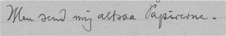Men send mig altsaa Papirerne.
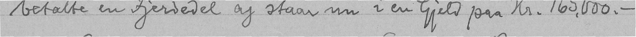betalte en Fjerdedel og staar nu i en Gjeld paa Kr. 165,000. -
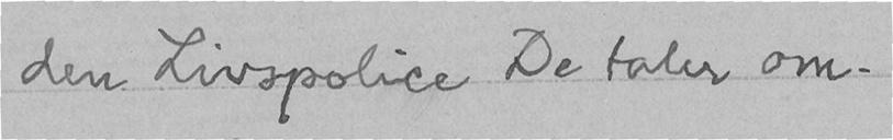den Livspolice De taler om.
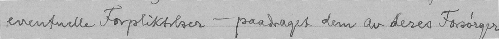eventuelle Forpliktelser - paadraget dem av deres Forsørger
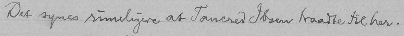Det synes rimeligere at Tancred Ibsen traadte til her.
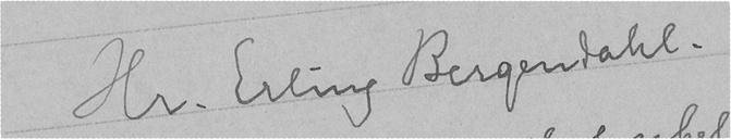Hr. Erling Bergendahl.
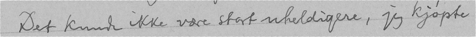Det kunde ikke være stort uheldigere, jeg kjøpte
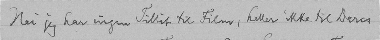Nei jeg har ingen Tillit til Film, heller ikke til Deres
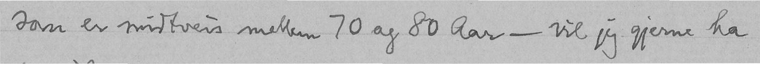som er midtveis mellem 70 og 80 Aar - vil jeg gjerne ha
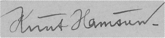Knut Hamsun.
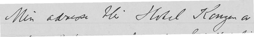Min adresse blir Hotel Kongen av
